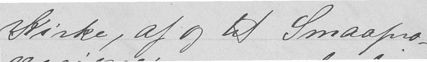Kirke, af og til Smaapro-
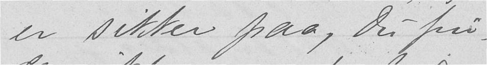er sikker paa, Du fin-
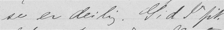se er deilig. Gid I fik
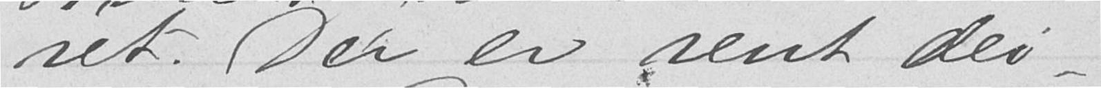ret. Der er rent dei-
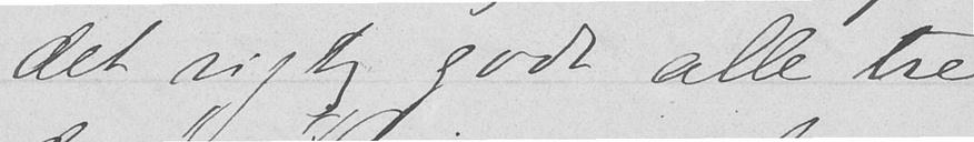det rigtig godt alle tre
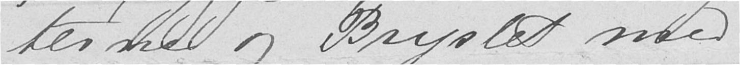terne og Brystet med
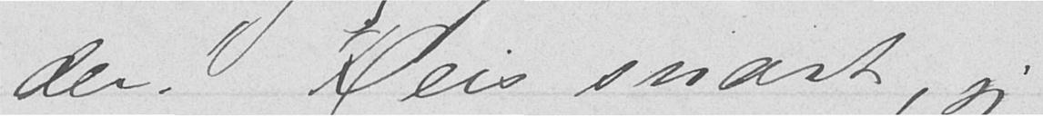der. Reis snart, jeg
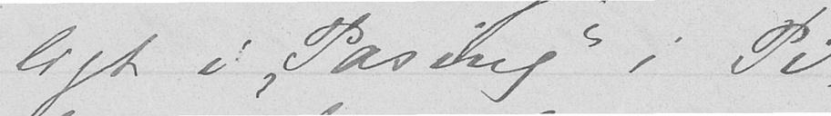ligt i "Pasing" i Pi-
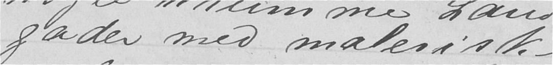gader med malerisk-
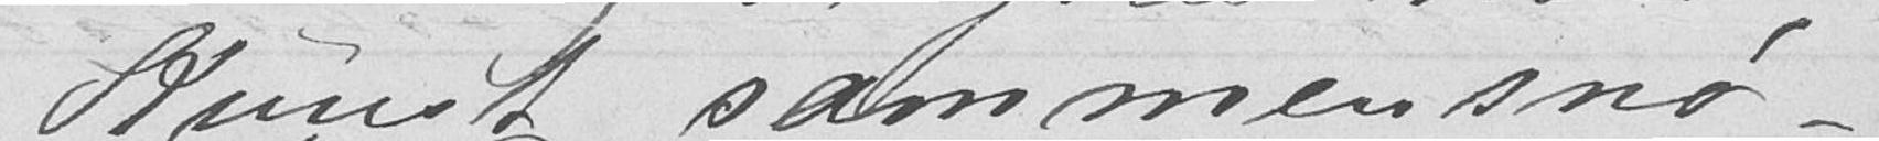Kunst sammensnø-
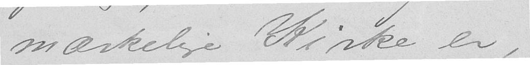mærkelige Kirke er,
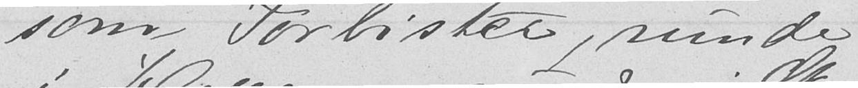som Forbister, runde
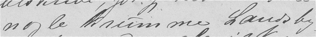nogle Krumme Landsby-
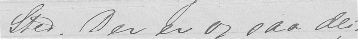Sted. Der er og saa dei-
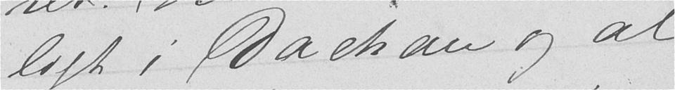ligt i Dachan og al
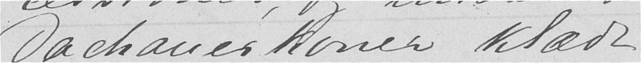Dachanerkoner klædt
